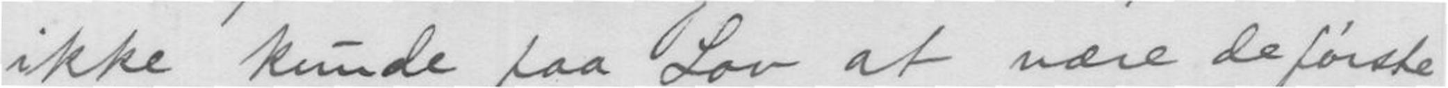ikke kunde faa Lov at være de første
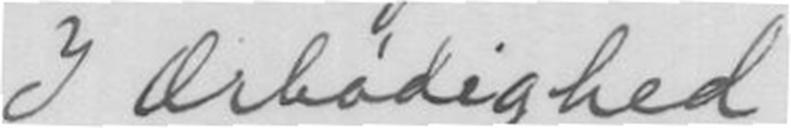I Ærbødighed
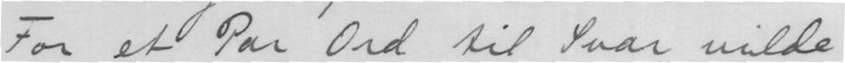For et Par Ord til Svar vilde
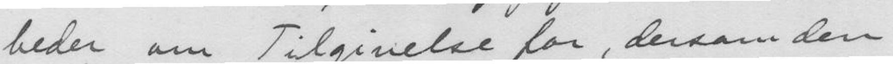beder om Tilgivelse for, dersom den
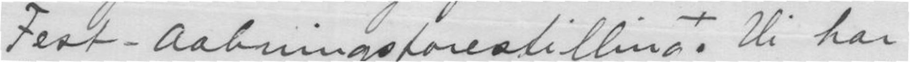Fest-Aabningsforestilling. Vi har
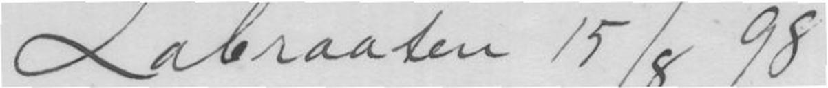Labraaten 15/8 98
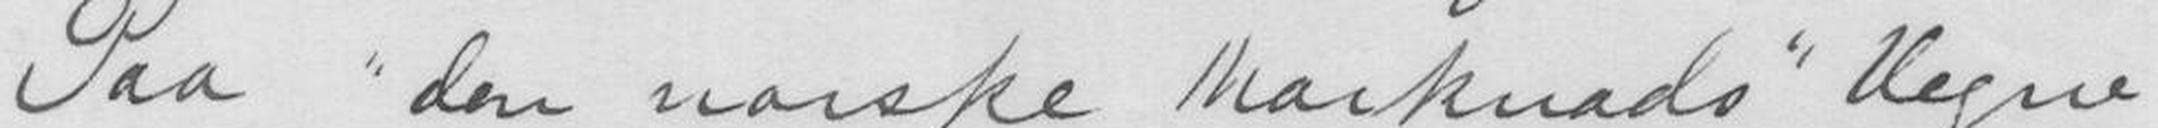Paa "den norske marknads" Vegne
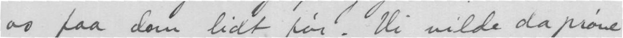os faa dem lidt før. Vi vilde da prøve
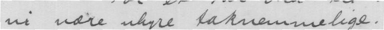vi være uhyre taknemmelige.
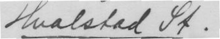Hvalstad St.
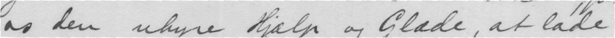os den uhyre Hjælp og Glæde, at lade
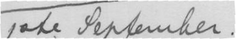1ste September.
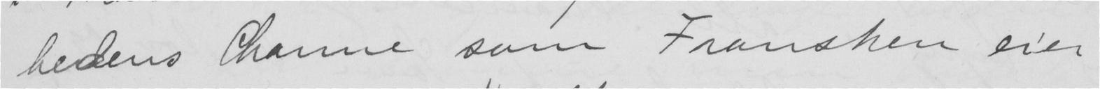hedens Charme som Fransken eier
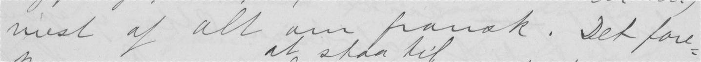mest af alt om fransk. Det fore-
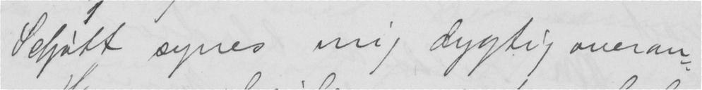Schjøtt synes mig dygtig overan-
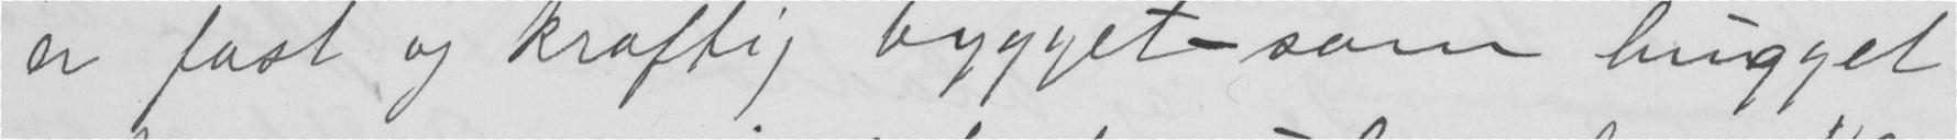er fast og kraftig bygget- som hugget
